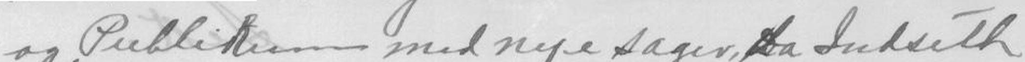og Publikum med nye sager, da Indseth
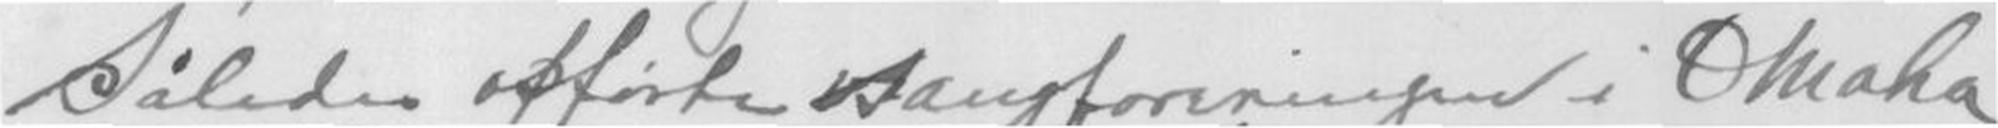Således opførte sangforeningen i Omaha
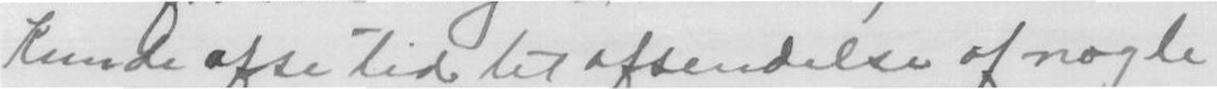kunde afse tid til afsendelse of nogle
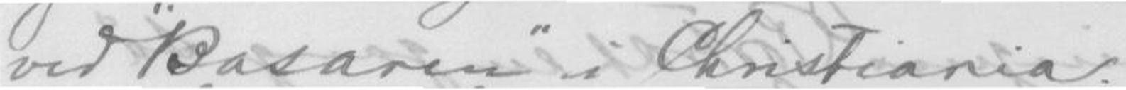ved "Basaren" i Christiania.
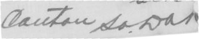Canton So Da
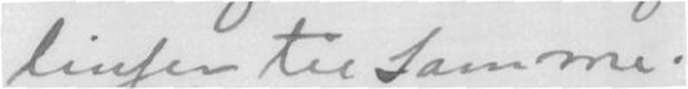linjer til samme.
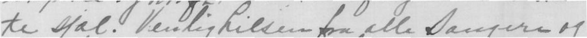te sjæl. Venlig hilsen fra alle Sangere og
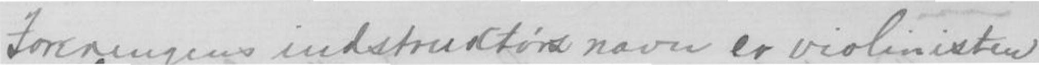Foreningens indstruktørs navn er violinisten
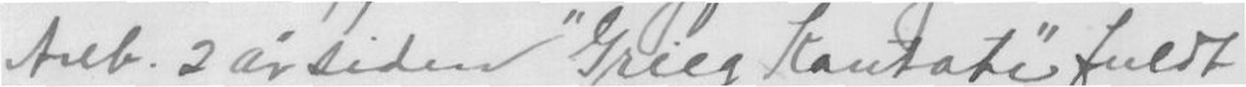Arlb. 2 År siden "Grieg Kantate" fuldt
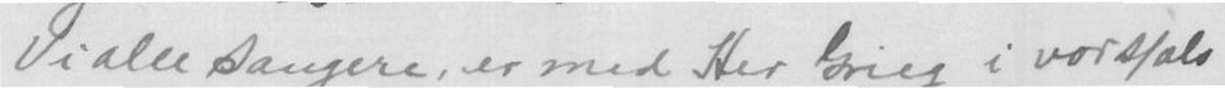Vi alle Sangere, er med Her Grieg i vor sjæls
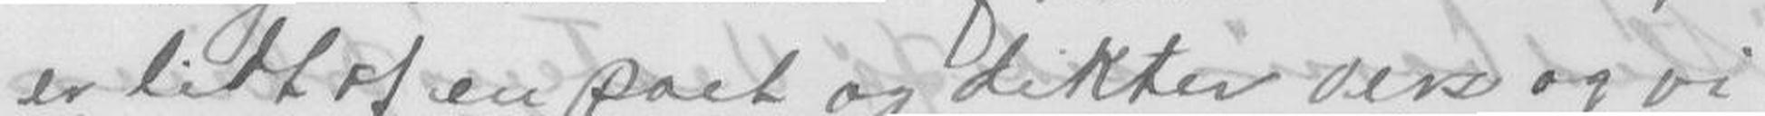er lidt af en poet og dikter vers og vi
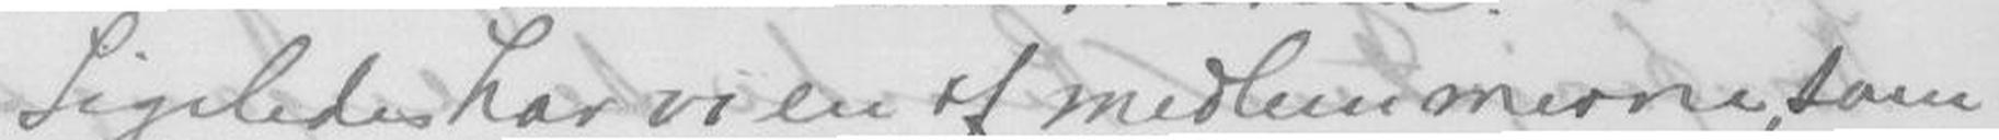Ligledes har vi en af medlemmerne, som
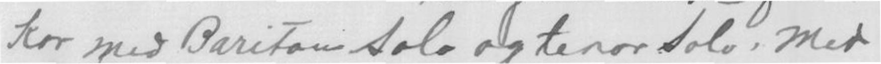kor med Bariton Solo og tenor Solo. Med
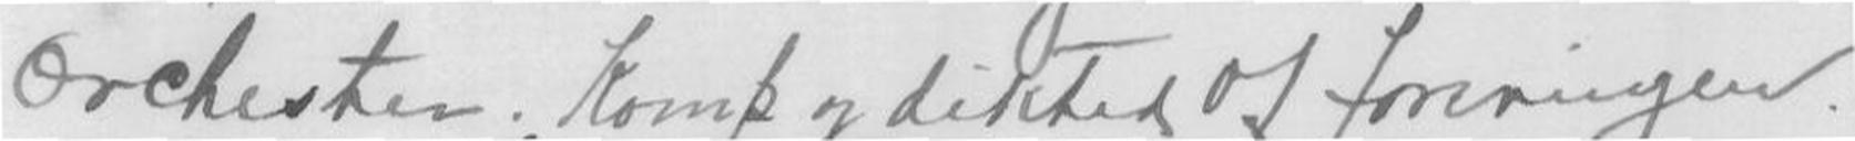Orchester. Komp og dikted of foreningen.
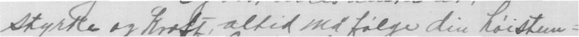styrke og Kraft, altid må følge din høistem-
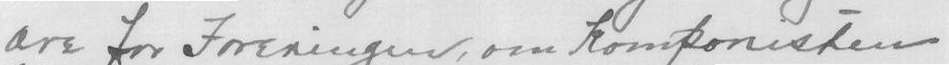Ære for Foreningen, om Komponisten
